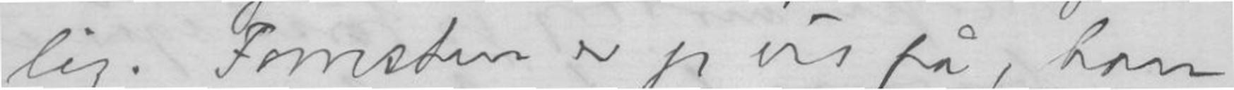lig. Forresten er jeg vis på, ham
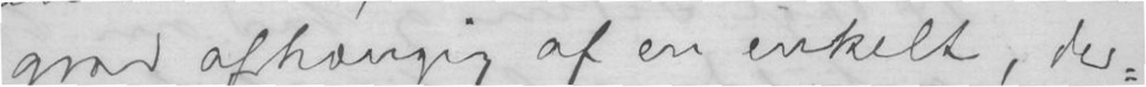grad afhængig af en enkelt, der-
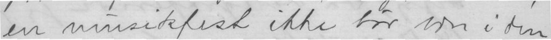en musikfest ikke bør være iden
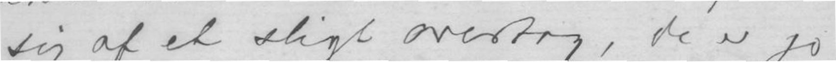sig af et slikt overtag, de er jo
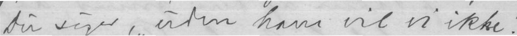Du siger, "uden ham vil vi ikke".
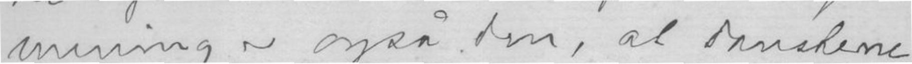mening er også den, at danskene
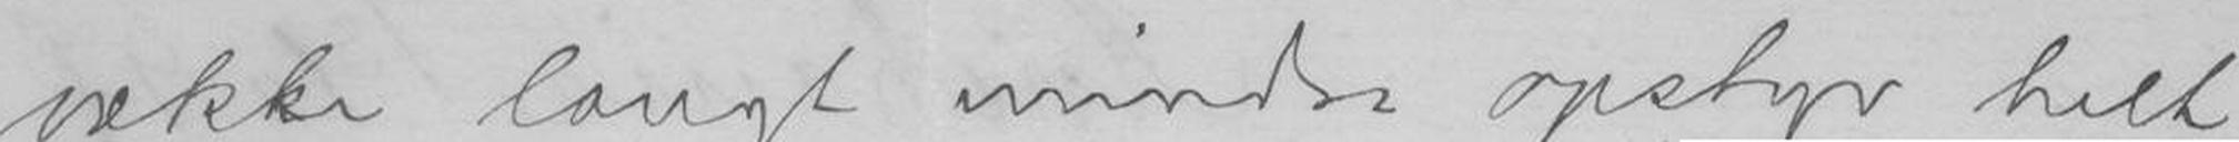vække langt mindre opstyr helt
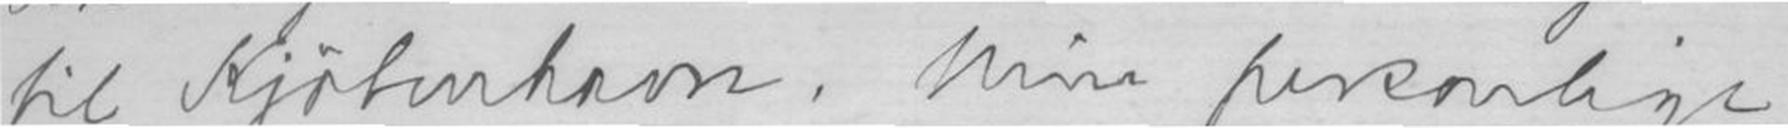til Kjøbenhavn. Min personlige
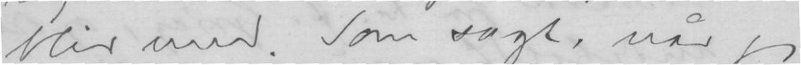blir med. Som sagt, når jeg
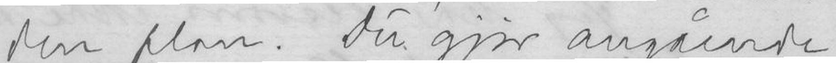den plan. Du gjør angående
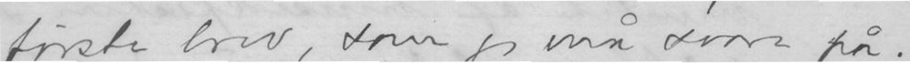første brev, som jeg må svare på.
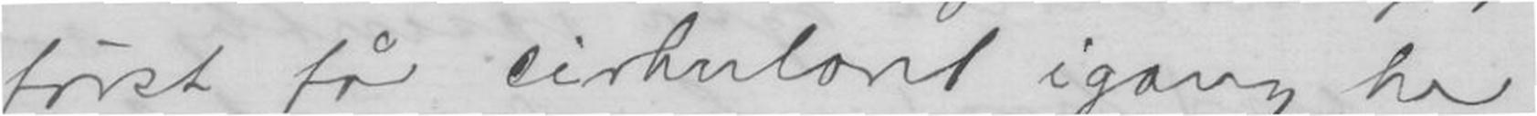først får cirkulæret igang her,
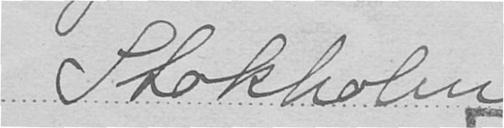Stokholm
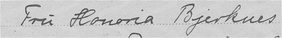Fru Honoria. Bjerknes
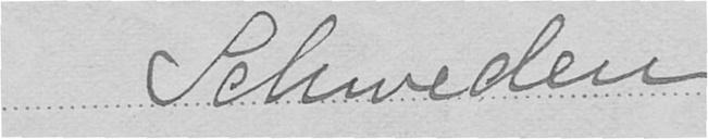Schweden
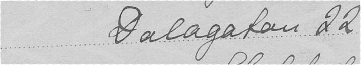Dalagatan 22
